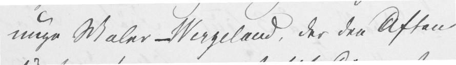unge Maler – Wergeland, der den Aften
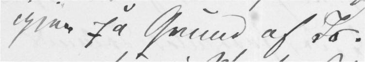igjen på Grund af Is.
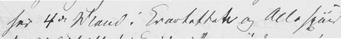her 4de Mand i Kvartetten og Alle synes
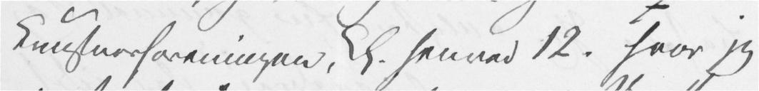Kunstnerforeningen, Kl. henved 12. hvor jeg
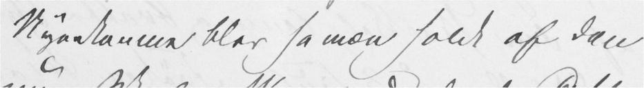Nyankommne blev såmæn holdt af den
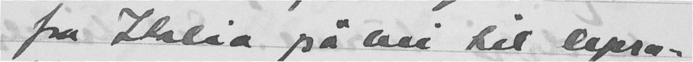fra Italia på vei til lepra-
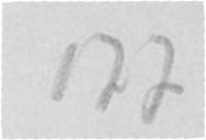177
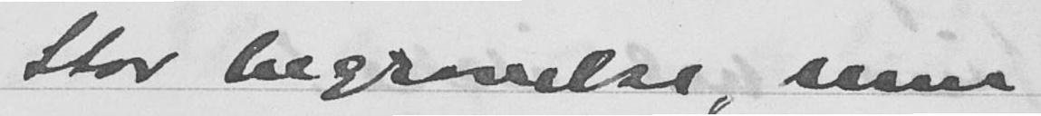Stor begravelse, men
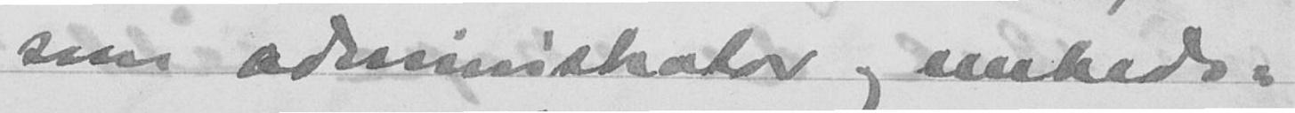som administrator og snkede-
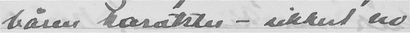båren karakter - sikkert en
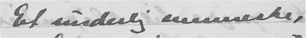Et underlig menneske,
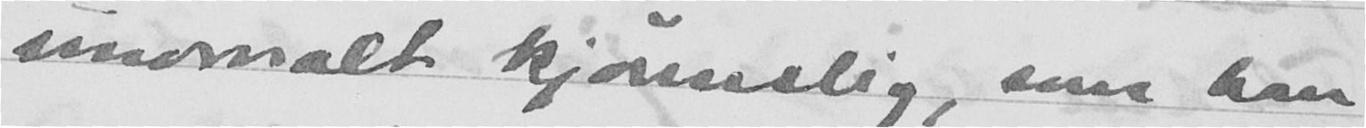unormalt kjønnslig, som han
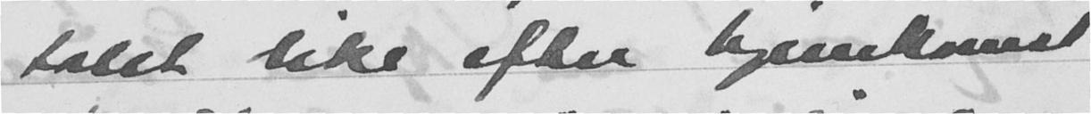talet like eftere hjemkomst
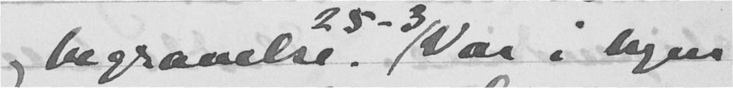og begravelse 25 - 3. Var i byen
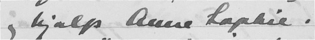og hjalp Anne Sophie.
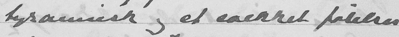tyrannisk og et svekket følelses-
